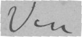Ven
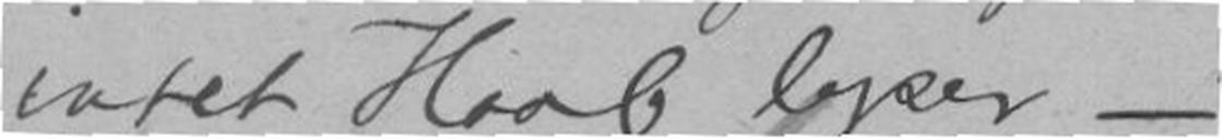intet Haab lyser -!
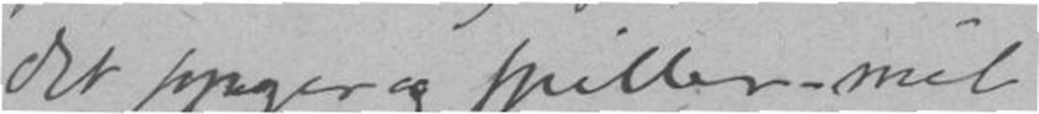det synger og spiller mit
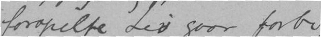forspilte Liv gaar forbi
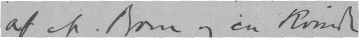af et Barn og en Kvinde,
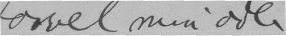Farvel min ædle
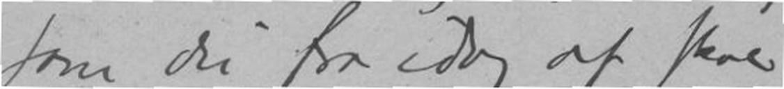som du fra idag af skal
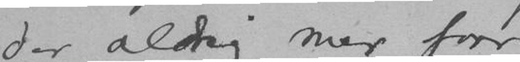der aldrig mer faar
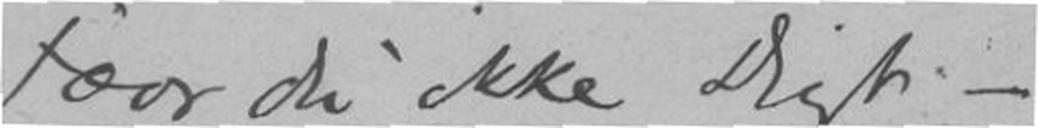Faar du ikke Digt -
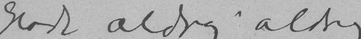glæde aldrig aldrig
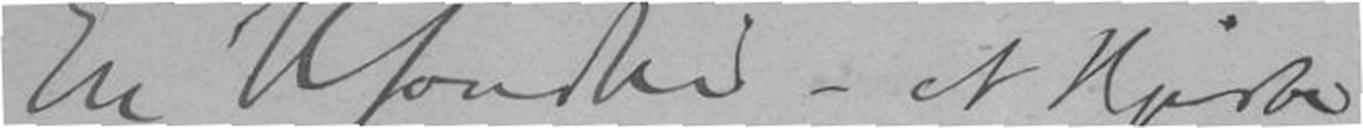En Usandhed i et Hjerte,
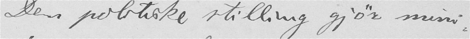Den politiske stilling gjør mini-
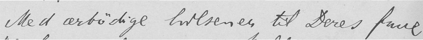Med ærbødige hilsener til Deres frue
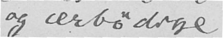og ærbødige
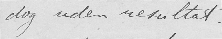dog uden resultat.
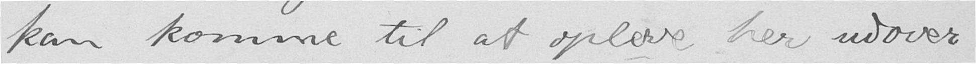kan komme til at opleve her udover
